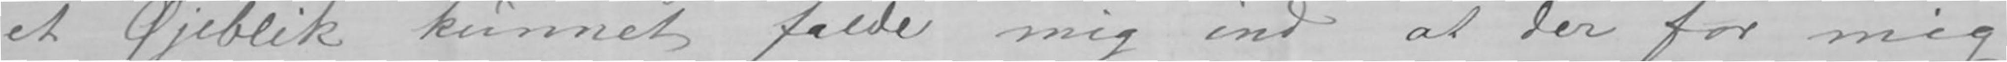et Øjeblik kunnet falde mig ind at der for mig
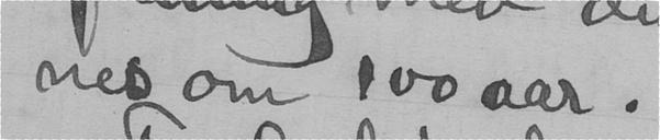nes om 100 aar.
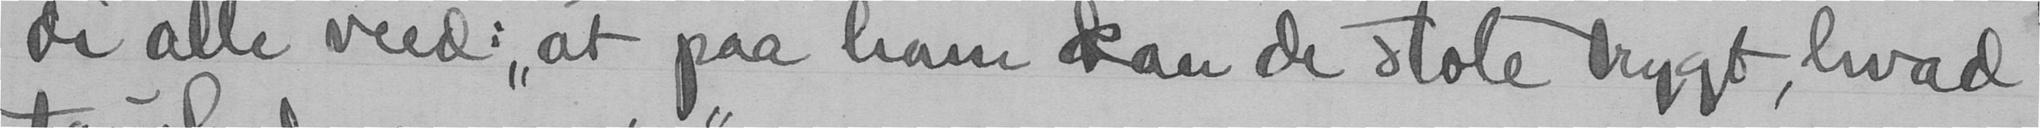di alle veed: "at paa ham kan de stole trygt, hvad
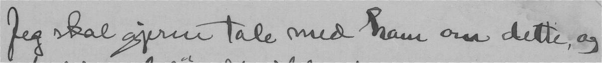Jeg skal gjerne tale med ham om dette, og
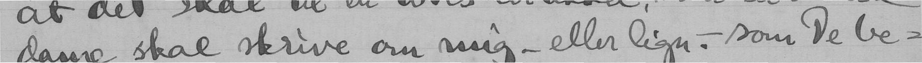dame skal skrive om mig - eller lign.- som De be-
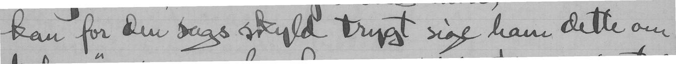kan for den sags skyld trygt sige ham dette om
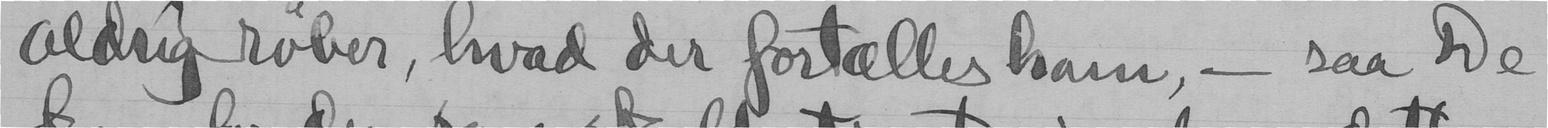aldrig røber, hvad der fortælles ham, - saa De
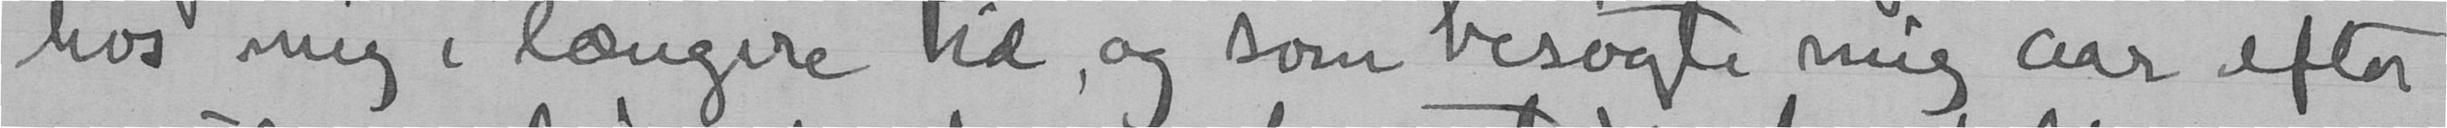hos mig i længere tid, og som besøgte mig aar efter
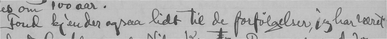Fond kjender ogsaa lidt til de forfølgelser, jeg har været
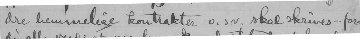dre hemmelige kontrakter o.s.v. skal skrives - for-
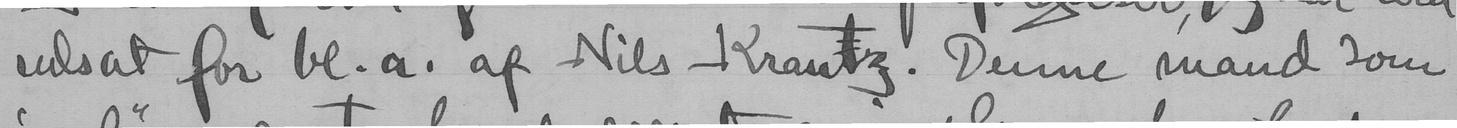udsat for bl. a. af Nils Kranz. Denne mand som
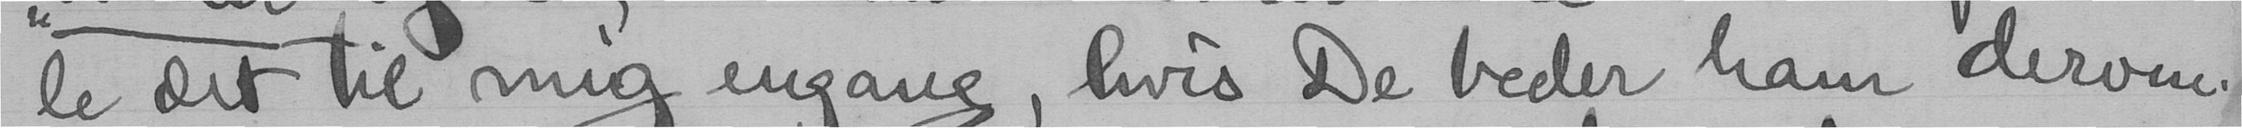te det til mig engang, hvis De beder ham derom.
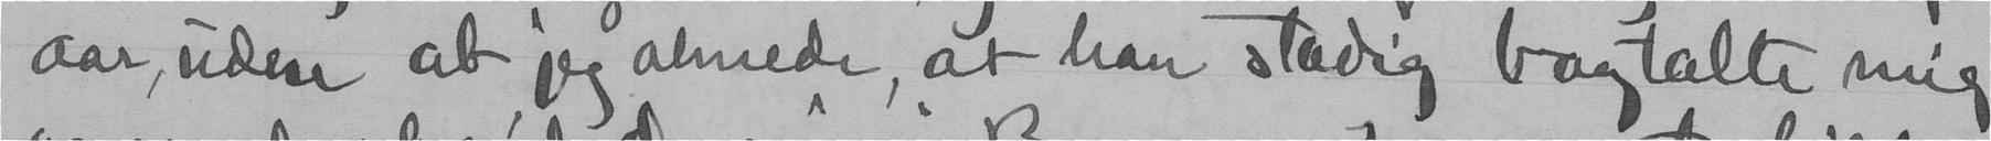aar, uden at jeg ahnede at han stadig bagtalte mig
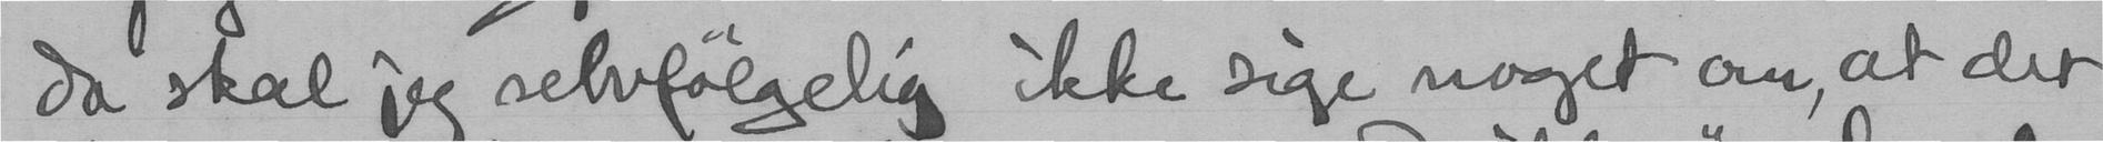da skal jeg selvfølgelig ikke sige noget om, at det
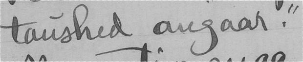taushed angaar".
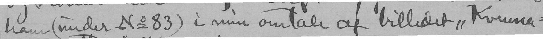ham (under No 83) i min omtale af billedet "Kvenna-
